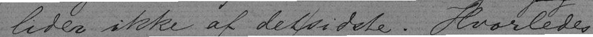lider ikke af det sidste. Hvorledes
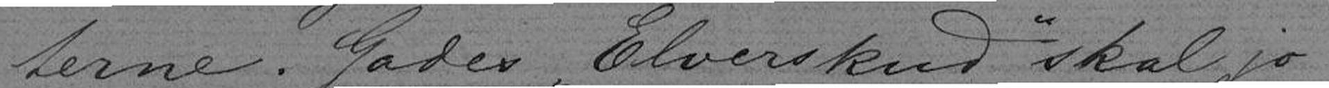terne. Gades Elverskud skal jo
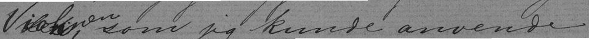overskrivning Violinen, som jeg kunde anvende
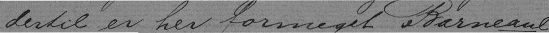dertil er her formeget Barneaul
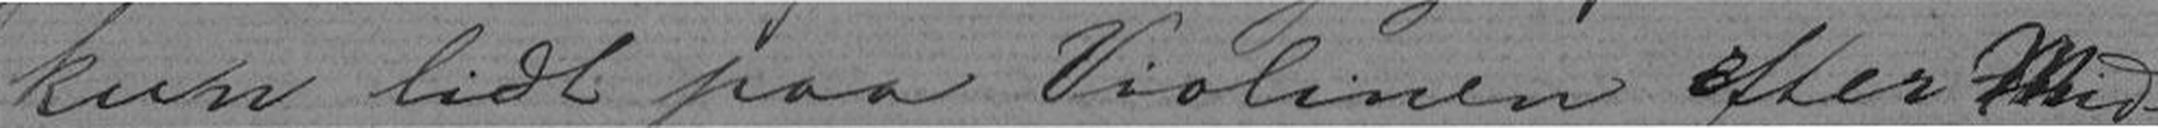kun lidt paa Violinen efter Mid-
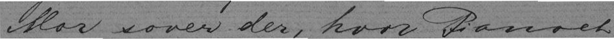Mor sover der, hvor Pianoet
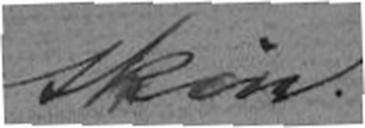skin.
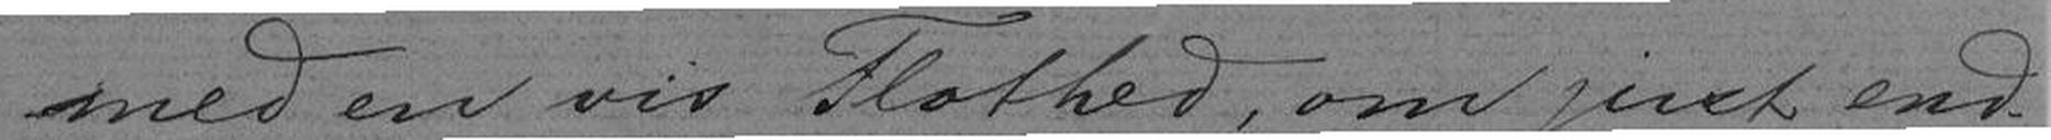med en vis Flothed, om just end-
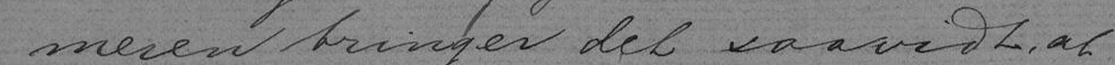meren bringer det saavidt, at
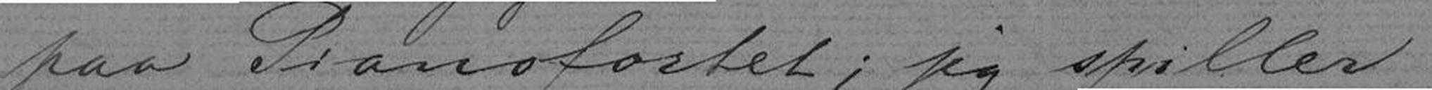paa Pianofortet; jeg spiller
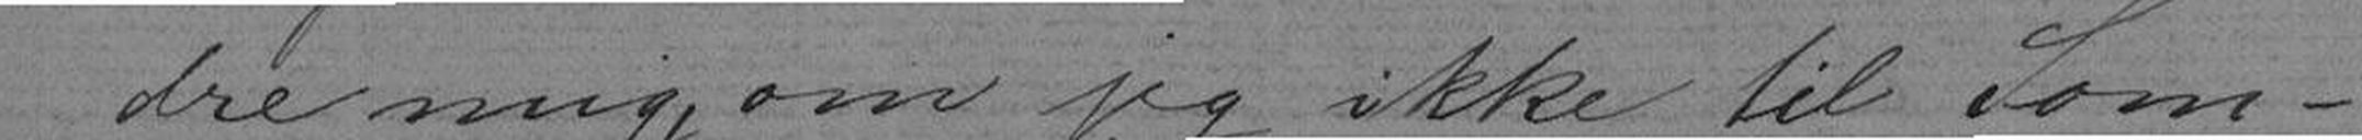dre mig om jeg ikke til Som-
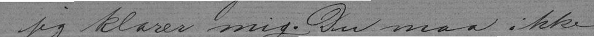jeg klarer mig. Du maa ikke
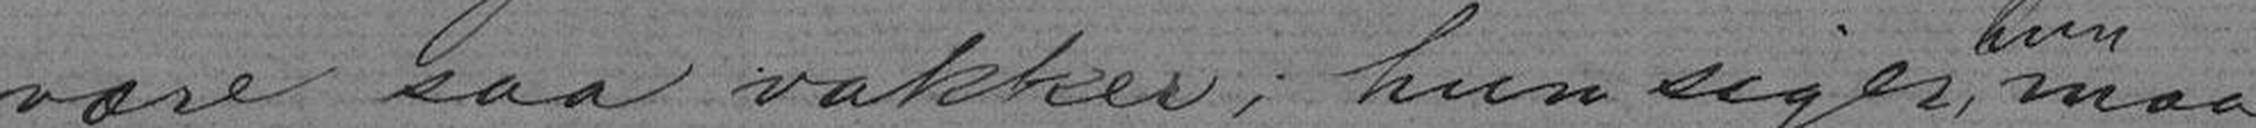være saa vakker; hun siger, maa
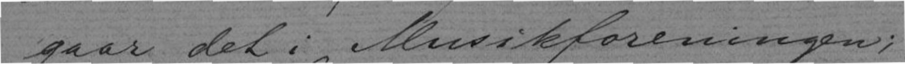gaar det i Musikforeningen;
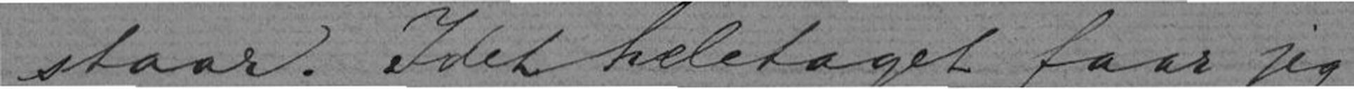staar. I det heletaget faar jeg
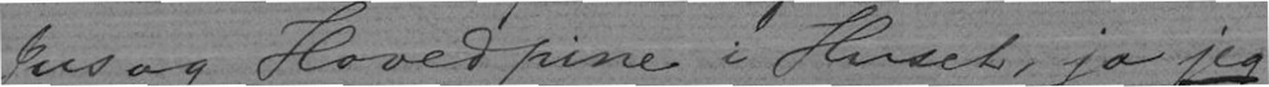Jus og Hovedpine i Huset, ja jeg
